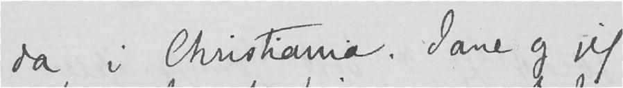da i Christiania. Jane og jeg
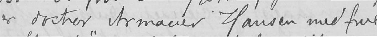er doctor Armauer Hansen med frue
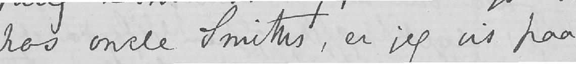hos oncle Smiths, er jeg vis paa,
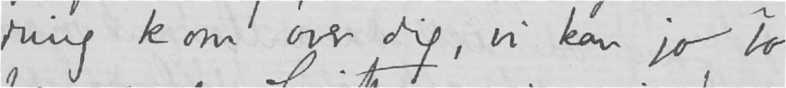dring kom over dig, vi kan jo bo
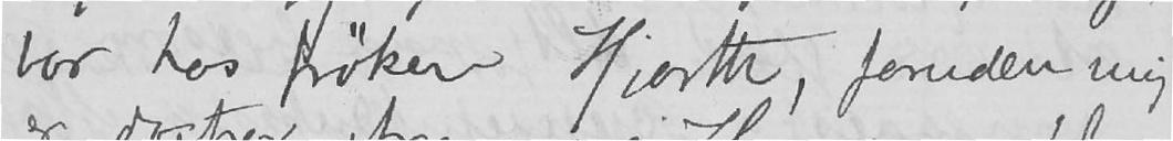bor hos frøken Hiorth, foruden mig
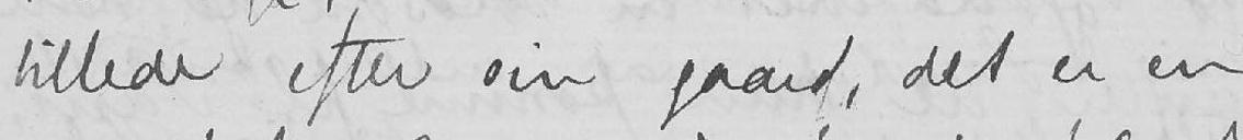billede efter sin gaard, det er en
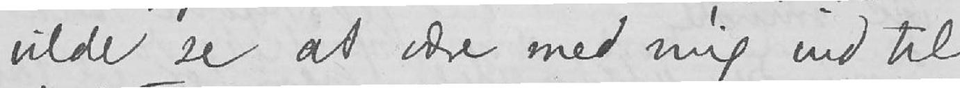vilde se at være med mig ind til
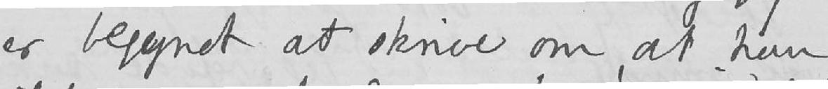er begyndt at skrive om, at hun
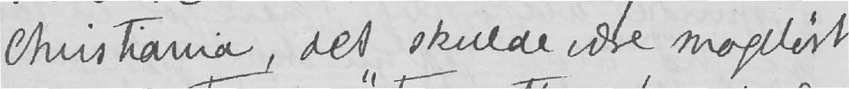Christiania, det skulde være mageløst
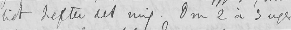lidt hefter det mig. Om 2 à 3 uger
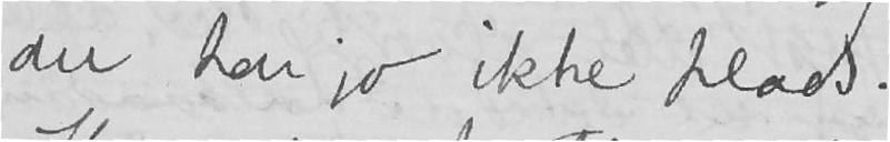du har jo ikke plads.
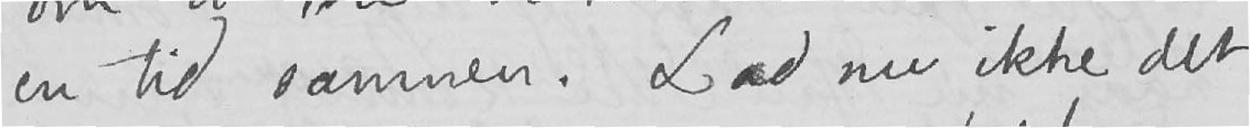en tid sammen. Lad nu ikke det
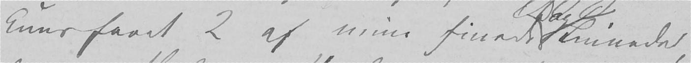kuns faaet 2 af mine finede Linneder
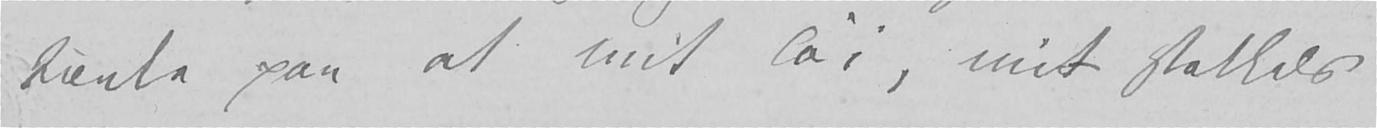tænke paa at mit Tøi, mit stakkels
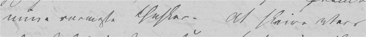mine varmeste Ønsker. At skrive Vers
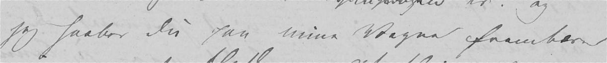jeg haaber Du paa mine Vegne frembærer
